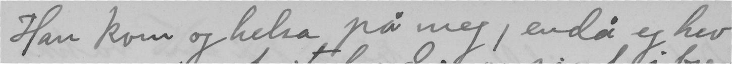Han kom og helsa på meg, endå eg hev
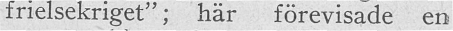frielsekriget"; här förevisade en
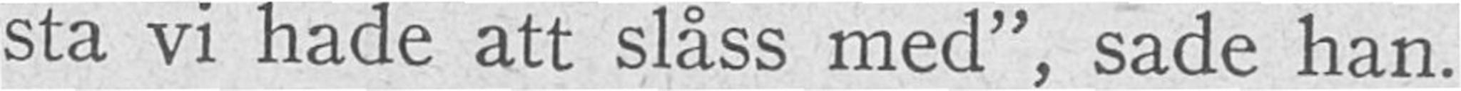sta vi hade att slåss med", sade han.
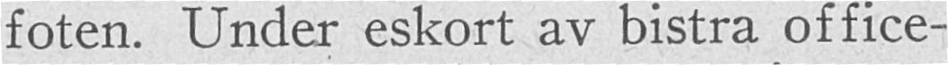foten. Under eskort av bistra office-
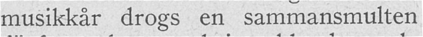musikkår drogs en sammansmulten
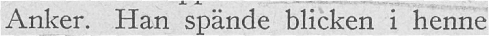Anker. Han spände blicken i henne
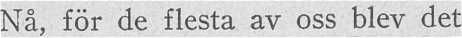- Nå, för de flesta av oss blev det
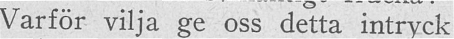Varför vilja ge oss detta intryck
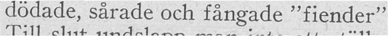dodade, sårade och fångade "fiender".
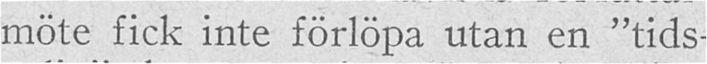möte fick inte förlöpa utan en "'tids-
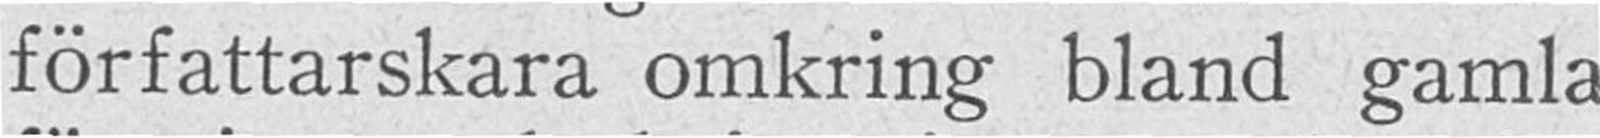författarskara omkring bland gamla
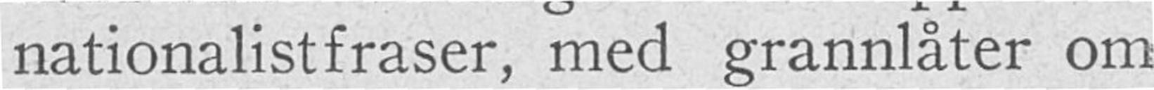nationalistfraser, med grannlåter om
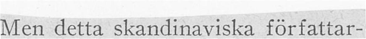Men detta skandinaviska författar-
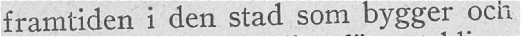framtiden i den stad som bygger och
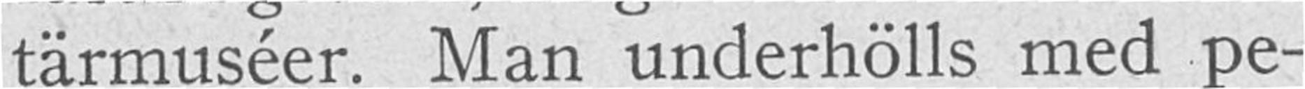tarmuséer. Man underhölls med pe-
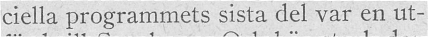ciella programmets sista del var en ut-
